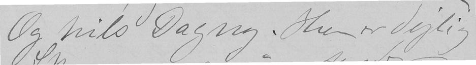Og hils Dagny. Hun er dejlig
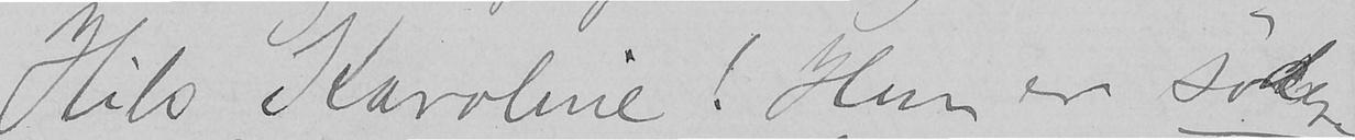Hils Karoline! Hun er sødere
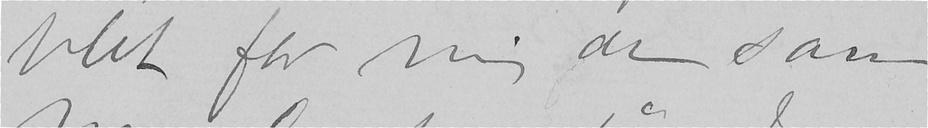blet for mig den sam-
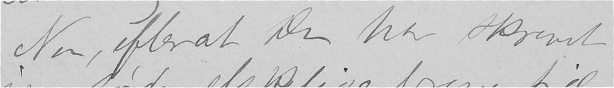Nu, efterat Du har skrevet
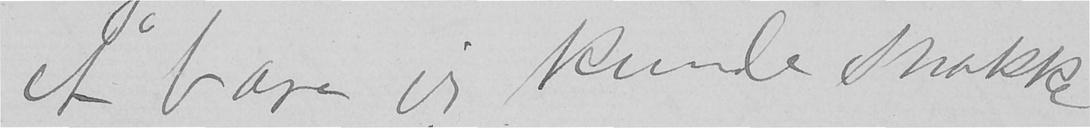Å bare jeg kunde snakke
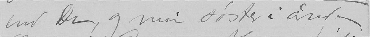end Du, og min søster i ånden.
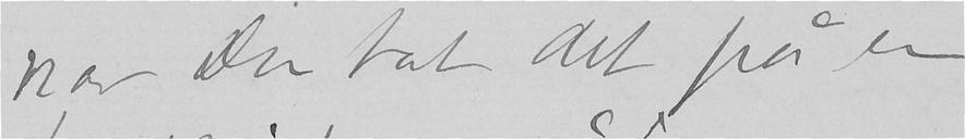har Du tat det på en
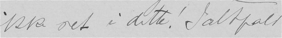ikke ret i dette! Ialtfald
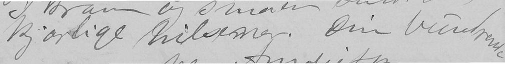kjærlige hilsener. Din beundrende
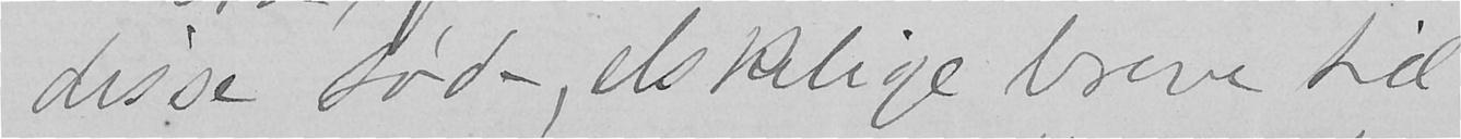disse søde, elskelige breve til
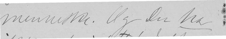menneske. Og Du har
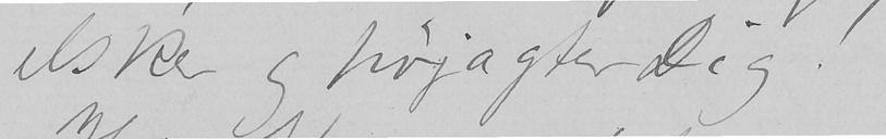elsker og højagter Dig!
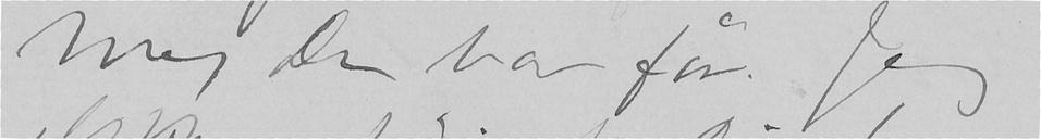me, Du var før. Jeg
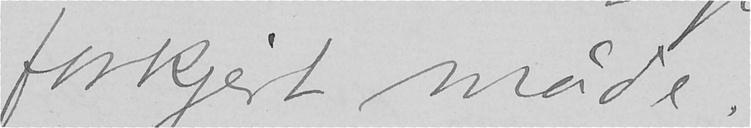forkjert måde.
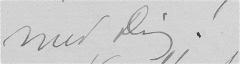med Dig!
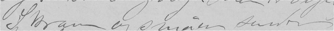Skram og småen sender
